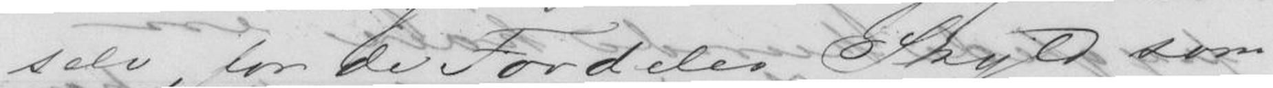selv, for de Fordeles Skyld som
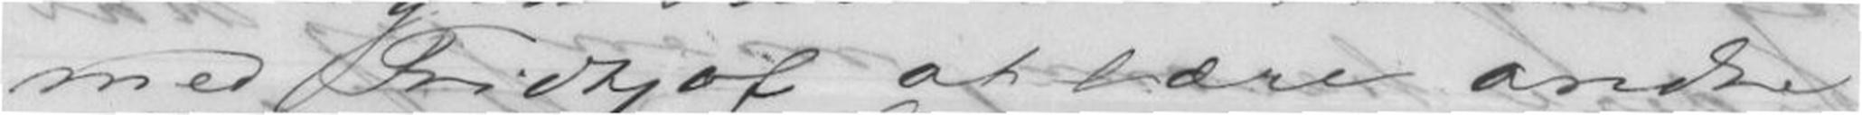med Fridtjof at lære andre
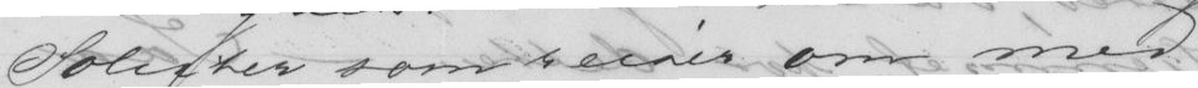Solister som reiser om med
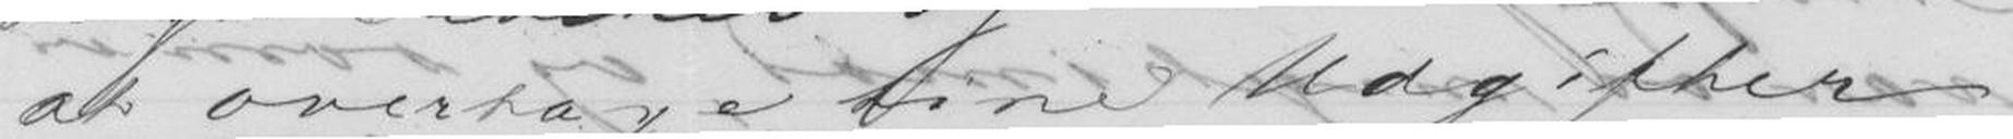at overtage sine Udgifter
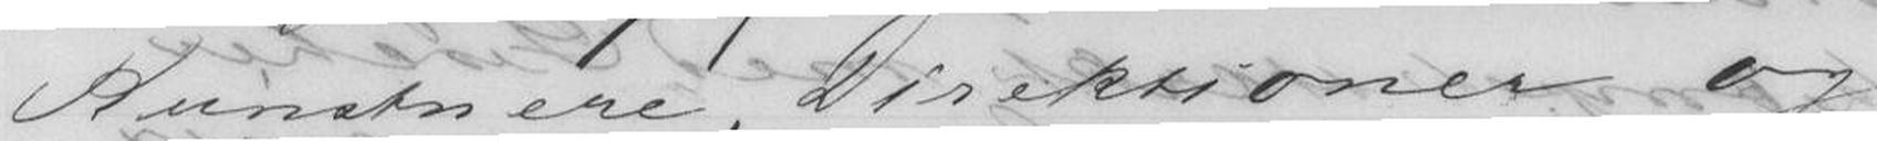Kunstnere, Direktioner og
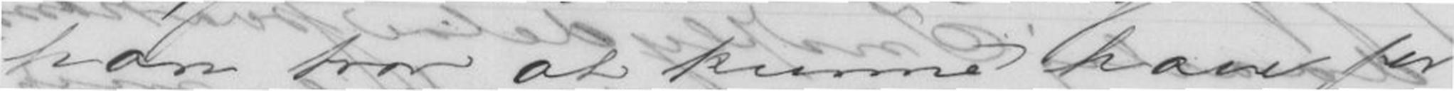han tror at kunne have for
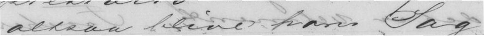altsaa blive hans Sag,
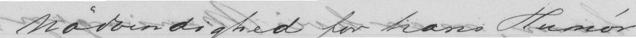Nødvendighed for hans Humør
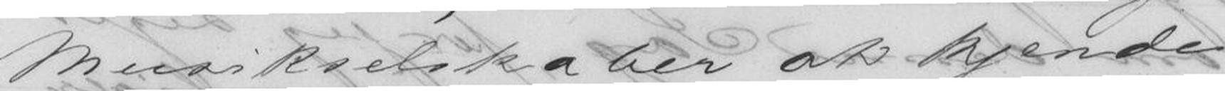Musikselskaber at kjende,
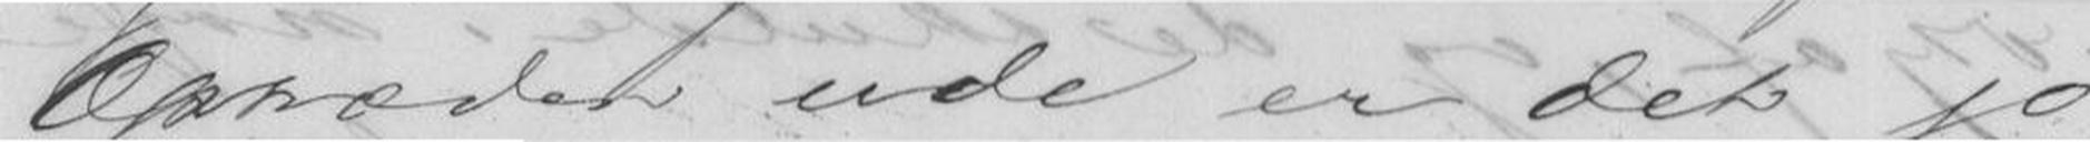Optræden ude er det jo
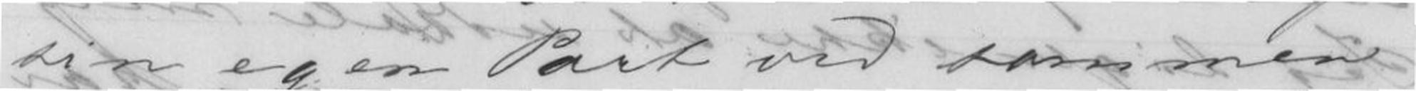sin egen Part ved sammen
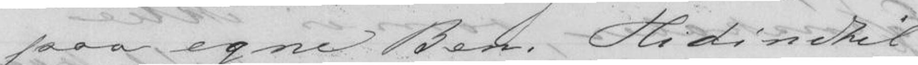paa egne Ben. Hidindtil
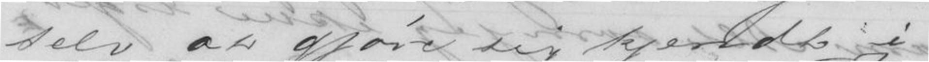selv at gjøre sig kjendt i
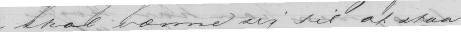skal vænne sig til at staa
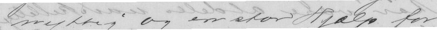nyttig og en stor Hjælp for
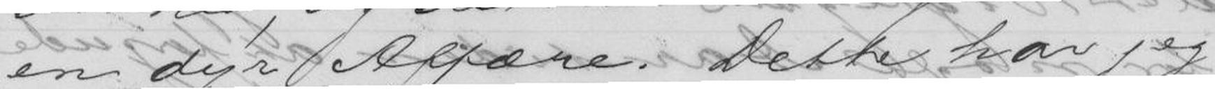en dyr Affære. Dette har jeg
